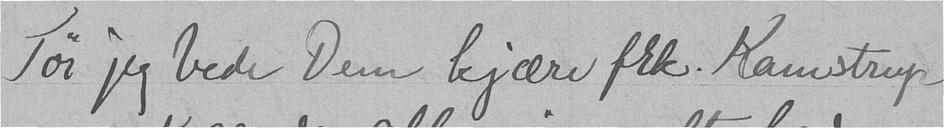Tør jeg bede Dem kjære frk. Kamstrup
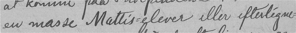en masse Mattis-elever eller efterligne-
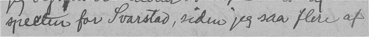spekten for Svarstad, siden jeg saa flere af
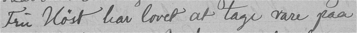Fru Høst har lovet at tage vare paa
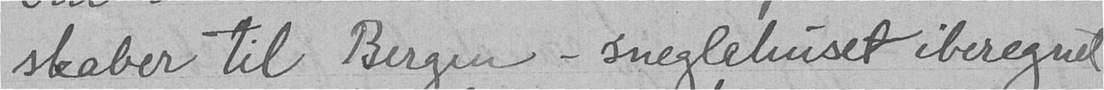skaber til Bergen - sneglehuset iberegnet
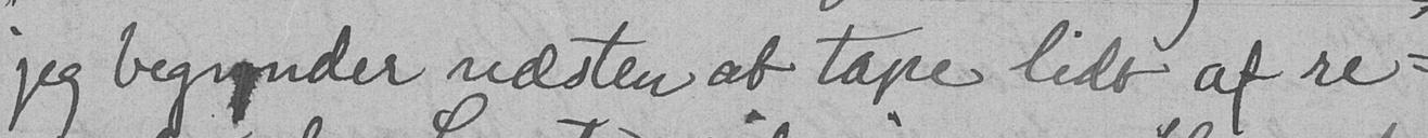jeg begynder næsten at tape lidt af re-
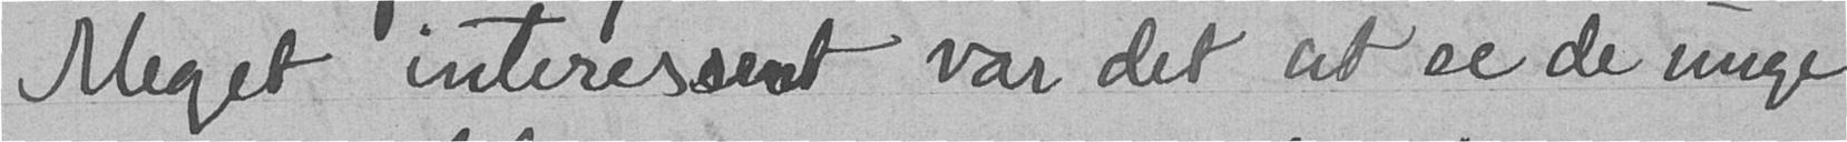Meget interessent var det at se de unge
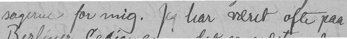sagerne for mig. Jeg har været ofte paa
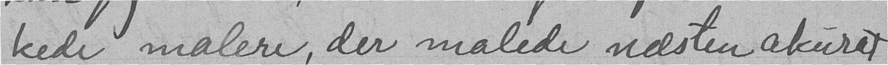kede malere, der malede næsten akurat
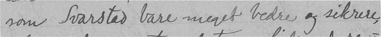som Svarstad bare meget bedre og sikrere,
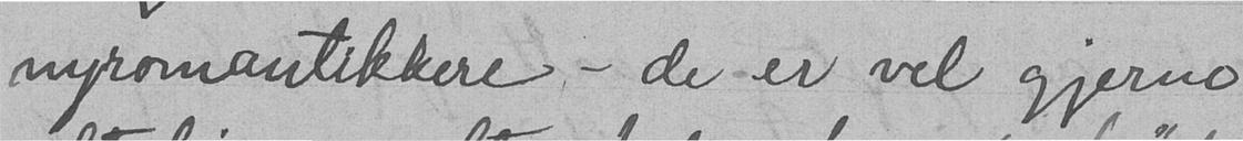nyromantikkere - de er vel gjerne
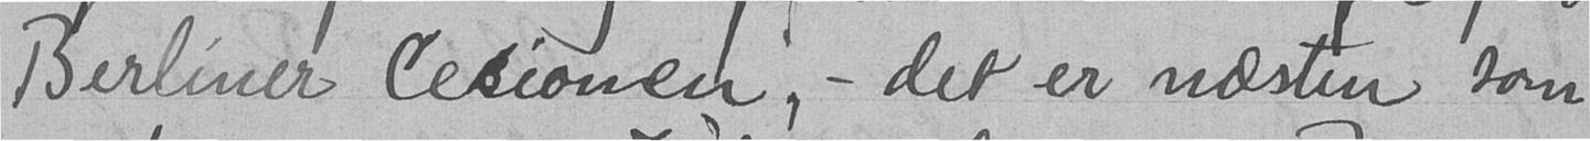Berliner cecionen, - det er næsten som
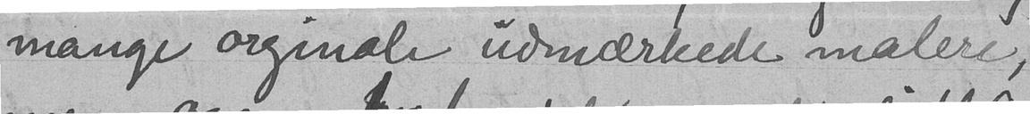mange orginale udmærkede malere,
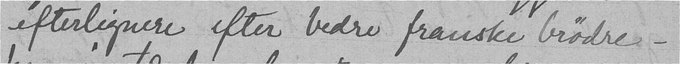efterlignere efter bedre franske brødre -
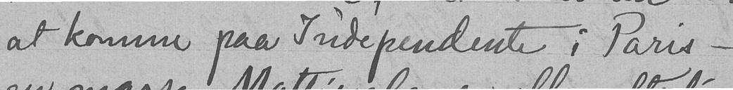at komme paa Independente i Paris -
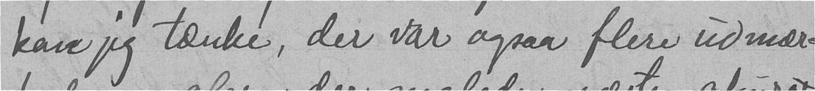kan jeg tænke, der var ogsaa flere udmær-
